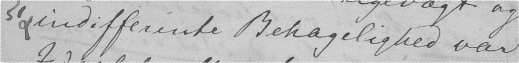indifferente Behagelighed var
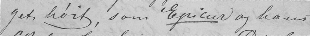get høit, som Epicur og hans
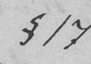§ 17
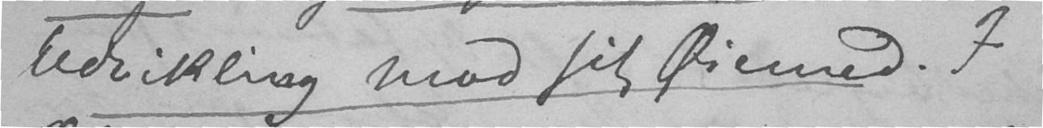udvikling mod sit Øiemed. I
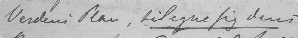Verdens Plan, tilegne sig dens
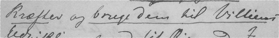Kræfter og bruge dem til Villiens
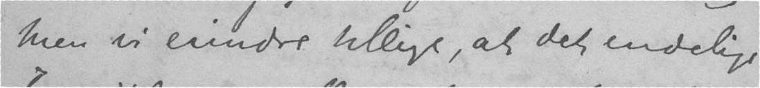Men vi erindre tillige, at det endelige
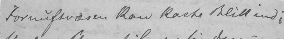Fornuftvæsen kan kaste Blik ind i
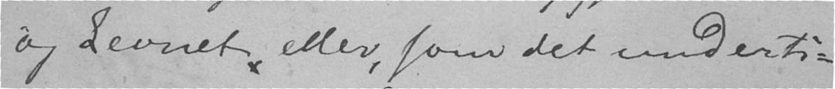og Levnet eller, som det underti-
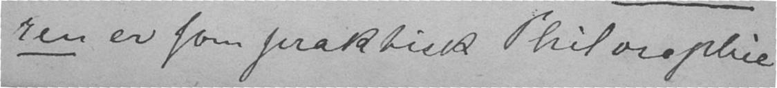ren er som praktisk Philosophie
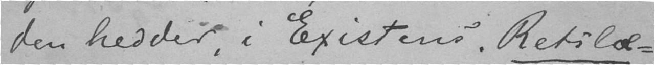den hedder, i Existens. Retslæ-
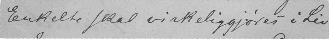Enkelte skal virkeliggjøres i Liv
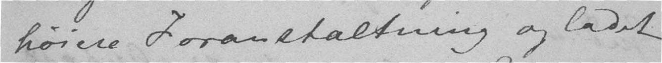høiere Foranstaltning og ladet
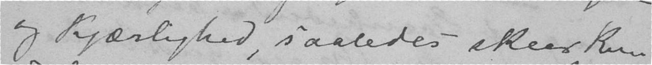og Kjærlighed, saaledes skeer kun
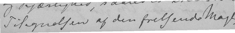Tilegnelsen af den frelsende Magt
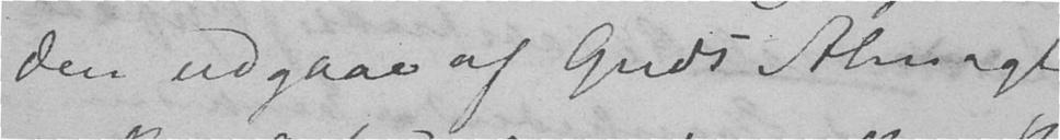den udgaae af Guds Almagt
